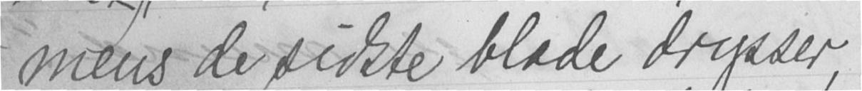mens de sidste blade drysser,
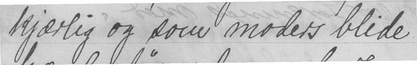kjærlig og som moders blide
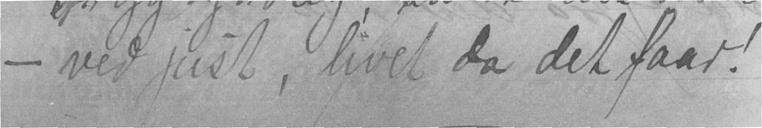- ved just, livet da det faar!
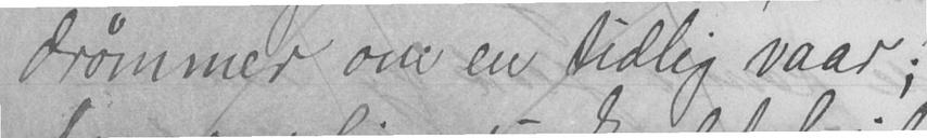drømmer om en tidlig vaar;
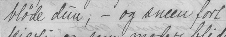bløde dun; - og sneen fort
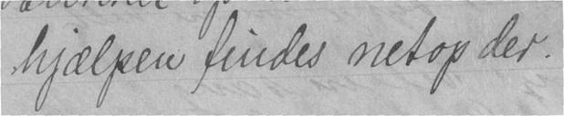hjælpen findes netop der.
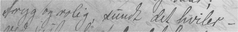tryg og rolig, sundt det hviler -
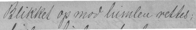Blikket op mod himlen rettes;
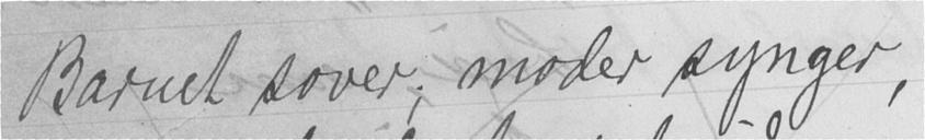Barnet sover, moder synger,
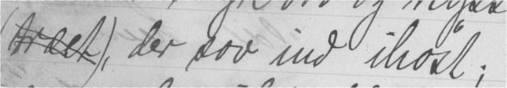(træet), der sov ind, ihøst;
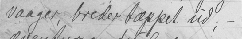vaager, breder tæppet ud; -
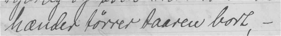hænder tørrer taaren bort, -
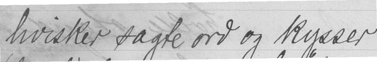hvisker sagte ord og kysser
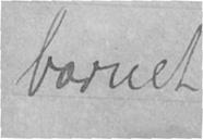barnet
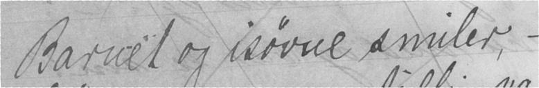Barnet og isøvne smiler, -
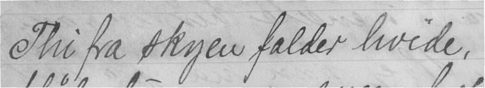Thi fra skyen falder hvide,
